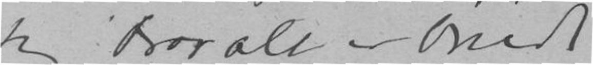jeg tror alt - Ordet
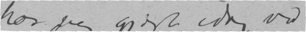har jeg gjort idag ved
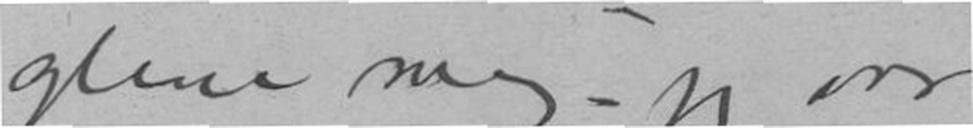glem mig - jeg var
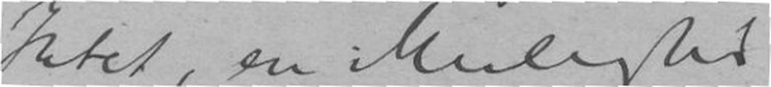Intet, en Mulighed
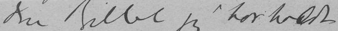den Billet jeg har holdt
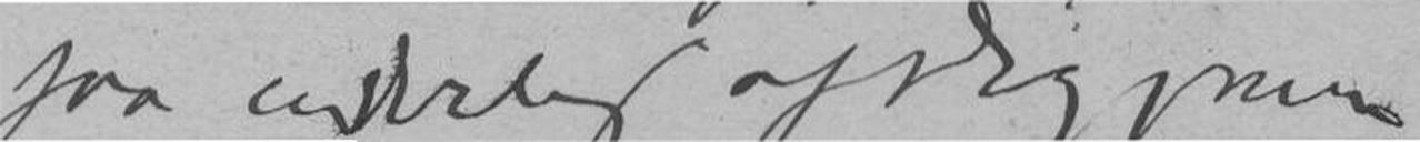saa inderlig af dig, men
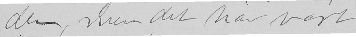den, men det har vært
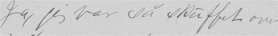Ja, jeg var så skuffet over
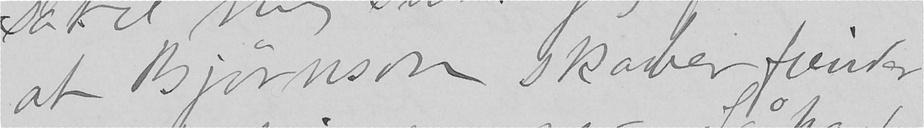at Bjørnson skaber fiender
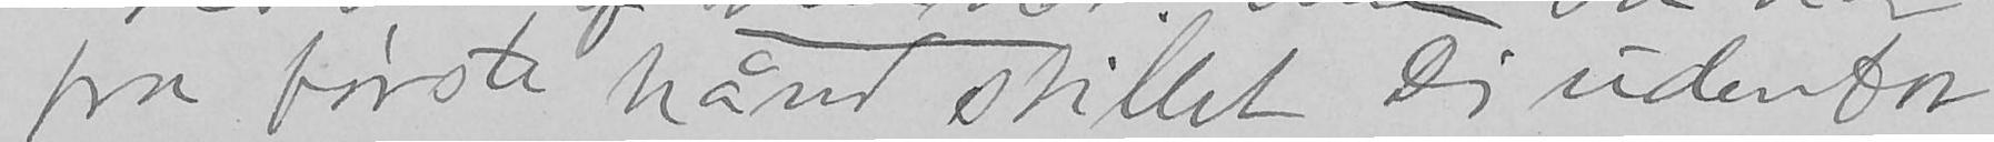fra første hånd stillet Dig udenfor
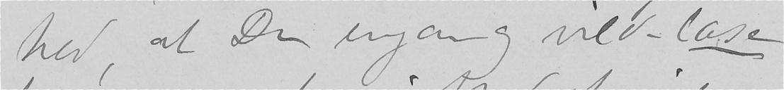hed, at Du engang vilde læse
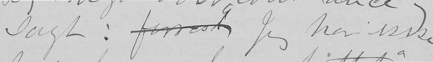sagt: forrest jeg har ikke
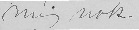mig nok.
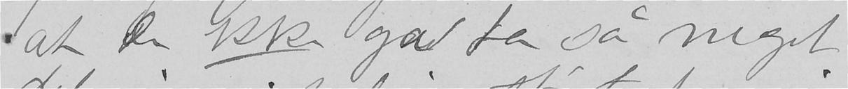at Du ikke gad ta så meget
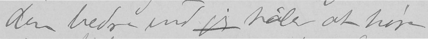den bedre end jeg tåler at høre
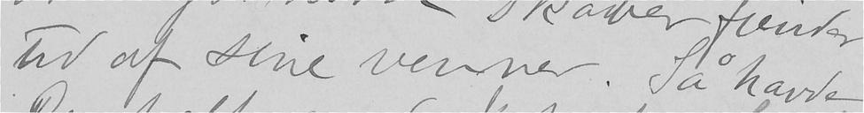ud af sine venner. Så havde
